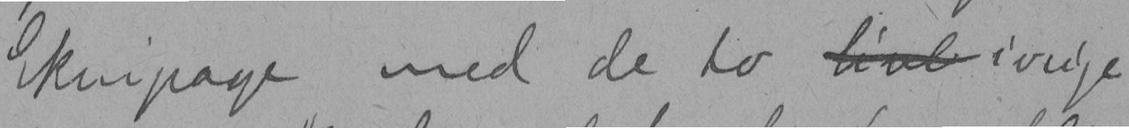Ekvipage med de to  ivrige
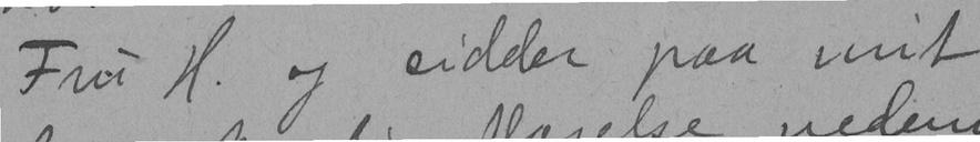Fru H. og sidder paa mit
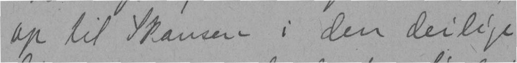op til Skansen i den deilige
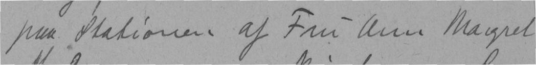paa Stationen af Fru Ann Margret
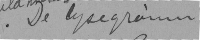. De lysegrønne
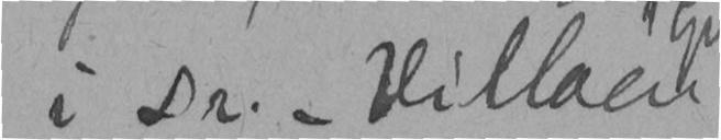i Dr. -Villaen
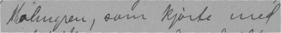Holmgren, som kjørte med
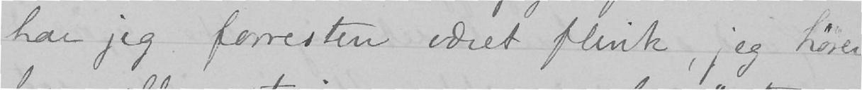har jeg forresten været flink, jeg hører
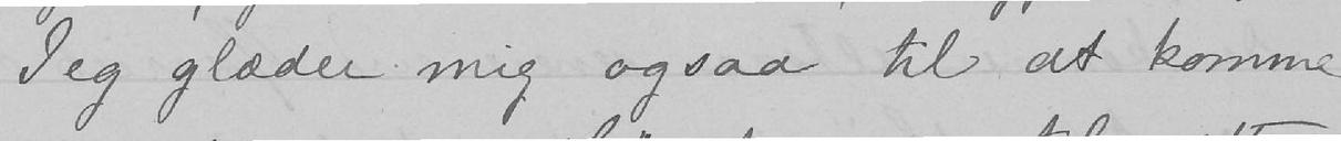Jeg glæder mig også til at komme
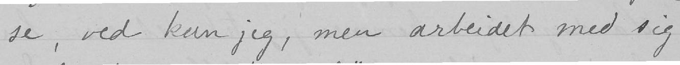se, ved kun jeg, men arbeidet med sig
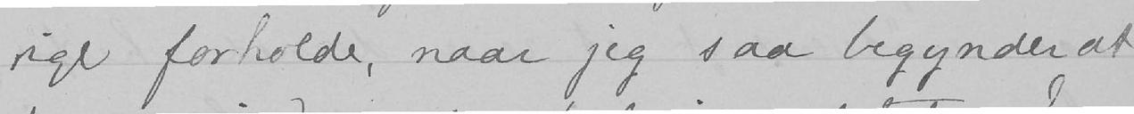rige forholde, naar jeg saa begynder at
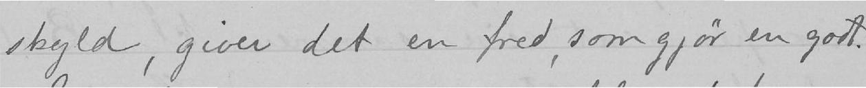skyld, giver det en fred, som gjør en godt.
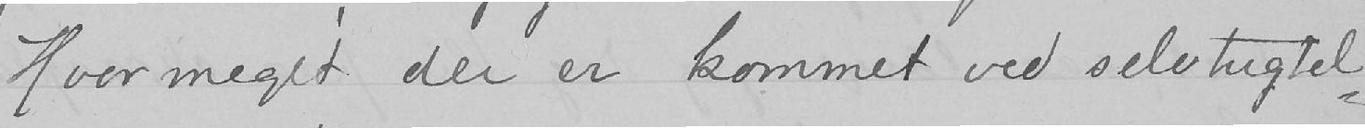Hvor meget der er kommet ved selvtugtel-
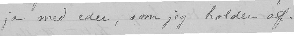ja med eder, som jeg holder af.
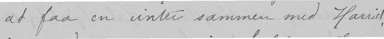at faa en vinter sammen med Harriet,
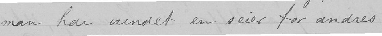man har vundet en seier for andres
